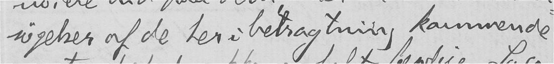søgelser af de her i betragtning kommende
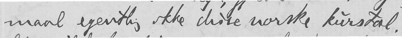maal egentlig ikke disse norske kurstal.
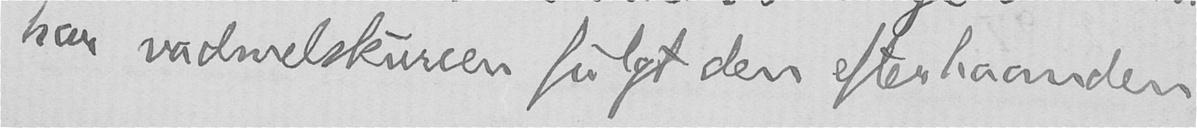har vadmelskursen fulgt den efterhaanden
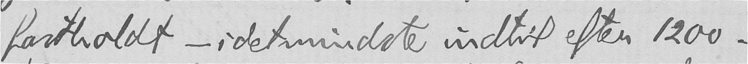fastholdt - idetmindste indtil efter 1200 -
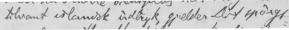tilvant islandsk udtryk, gjælder Dit spørgs-
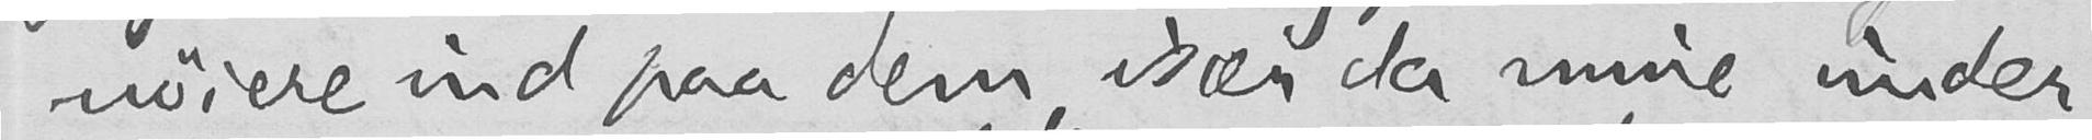nøiere ind paa dem, især da mine under-
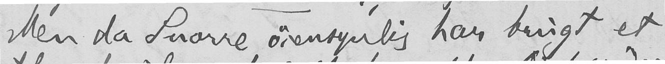Men da Snorre øiensynlig har brugt et
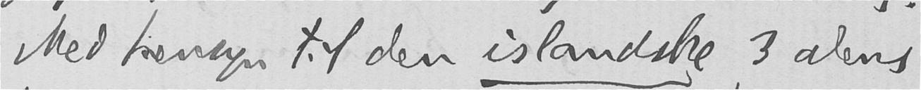Med hensyn til den islandske 3 alens
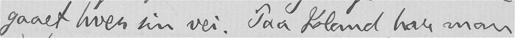gaaet hver sin vei. Paa Island har man
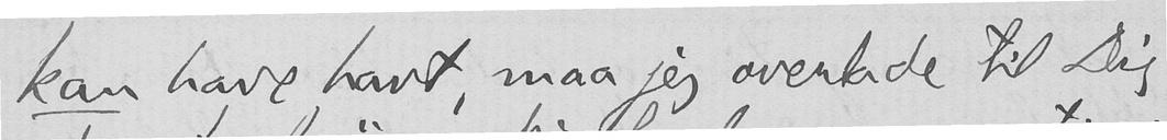kan have havt, maa jeg overlade til Dig
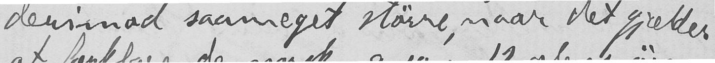derimod saameget større, naar det gjælder
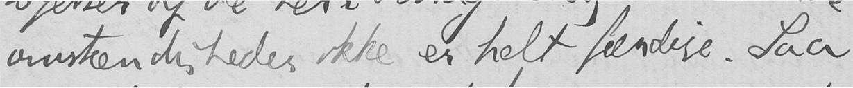omstændigheder ikke er helt færdige. Saa
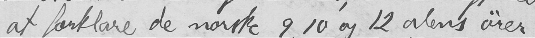at forklare de norske 9, 10 og 12 alens ører.
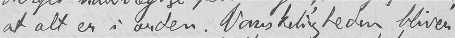at alt er i orden. Vanskeligheden bliver
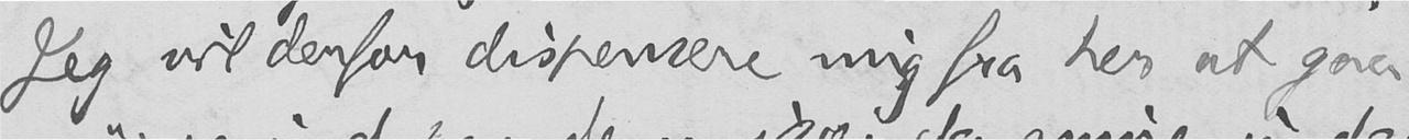Jeg vil derfor dispensere mig fra her at gaa
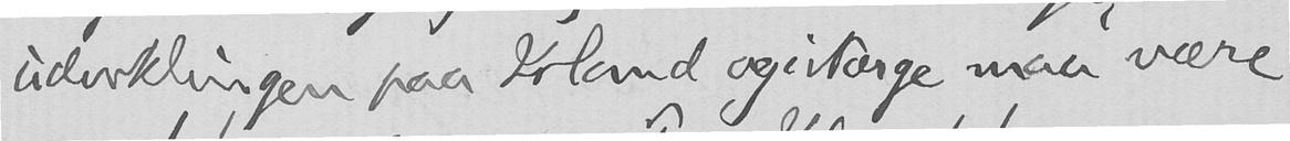udviklingen paa Island og Norge maa være
